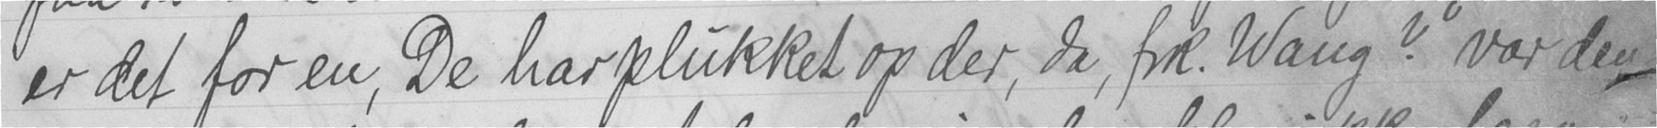er det for en, De har plukket op der, da, frk. Wang?" var den
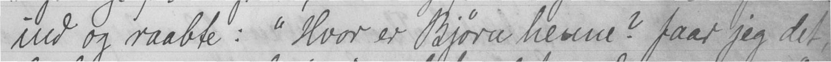ind og raabte: "Hvor er Bjørn henne? faar jeg det,
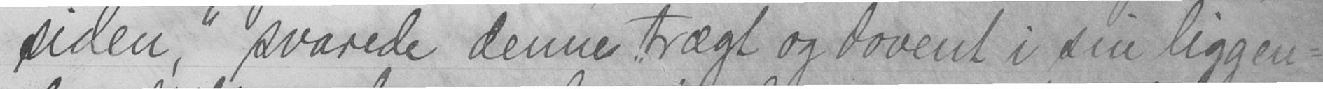siden," svarede denne trægt og dovent i sin liggen-
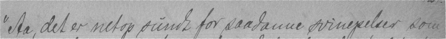"Aa, det er netop sundt for saadanne svinepelser som
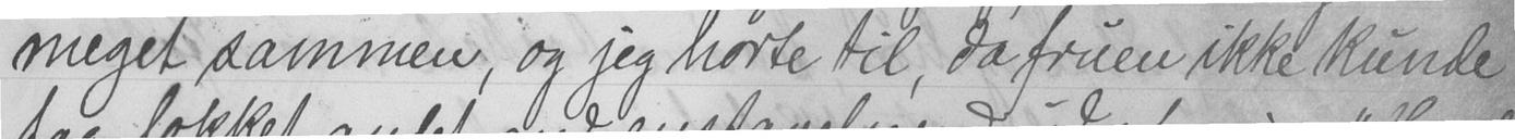meget sammen, og jeg hørte til, da fruen ikke kunde
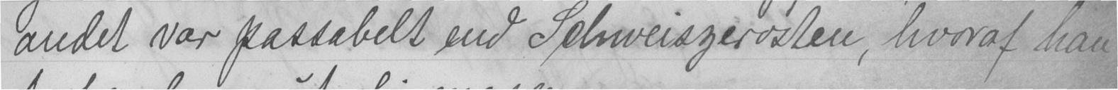andet var passabelt end Schweiszerosten, hvoraf han
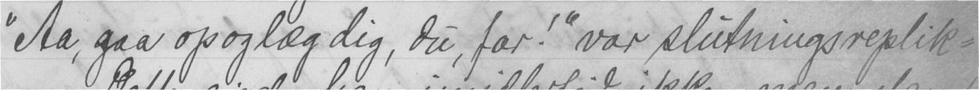"Aa, gaa op og læg dig, du, far!" var slutningsreplik-
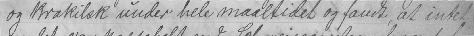og krakilsk under hele maaltidet og fandt, at intet
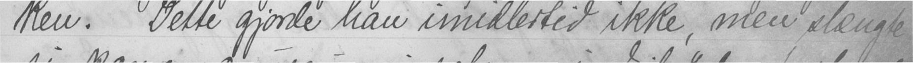ken. Dette gjorde han imidlertid ikke, men slængte
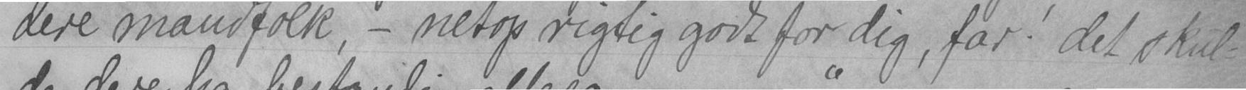dere mandfolk, - netop rigtig godt for dig, far! det skul-
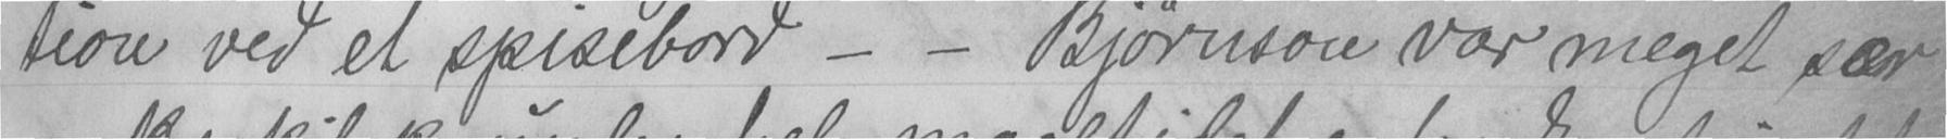tion ved et spisebord - - Bjørnson var meget sær
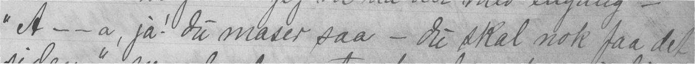"A -- a, ja! du maser saa - du skal nok faa det
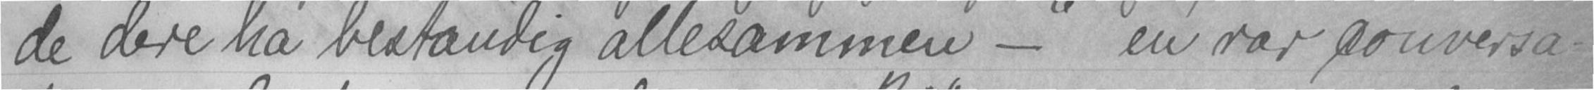de dere ha bestandig allesammen - " en rar conversa-
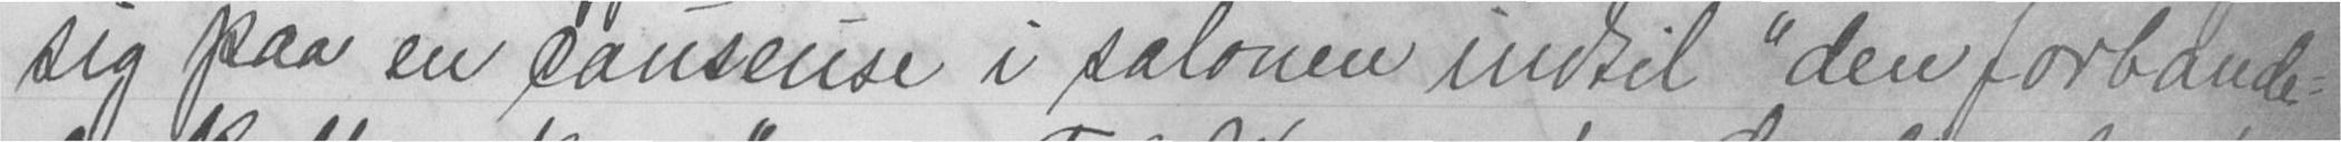sig paa en causeuse i salonen indtil "den forbande-
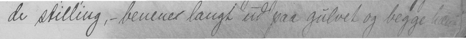de stilling, - benener langt ud paa gulvet og begge hæn-
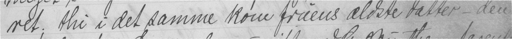ret; thi i det samme kom fruens ældste datter - den
